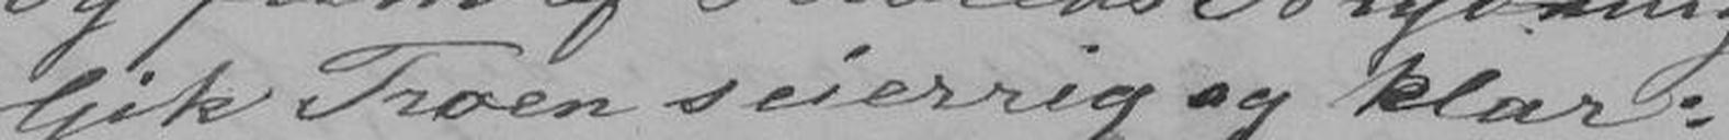Gik Troen seierrig og klar:
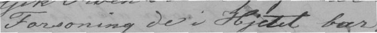Forsoning de i Hjertet bar,
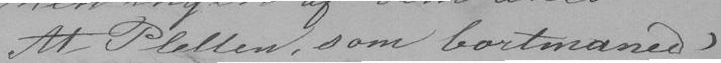At Pletten, som bortmaned'
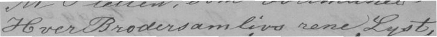Hver Brodersamlivs rene Lyst,
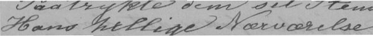Hans hellige Nærværelse
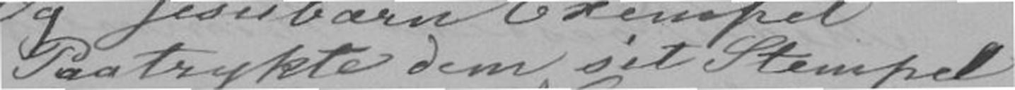Paatrygte dem sit Stempel
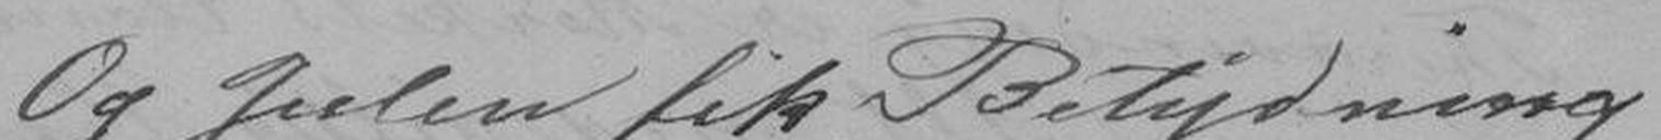Og Julen fik Betydning,
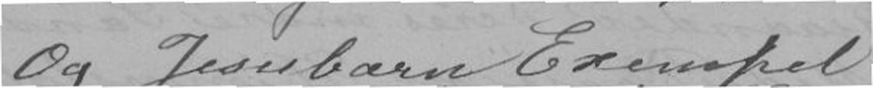Og Jesubarn Exempel
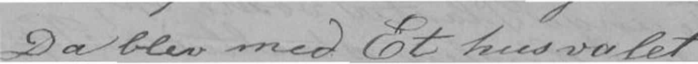Da blev med Et husvalet
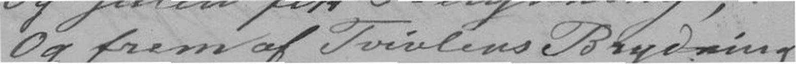Og frem af Tvilens Brydning
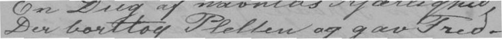Der borttog Pletten og gav Fred.
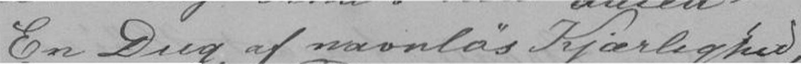En Dug af navnløs Kjærlighed,
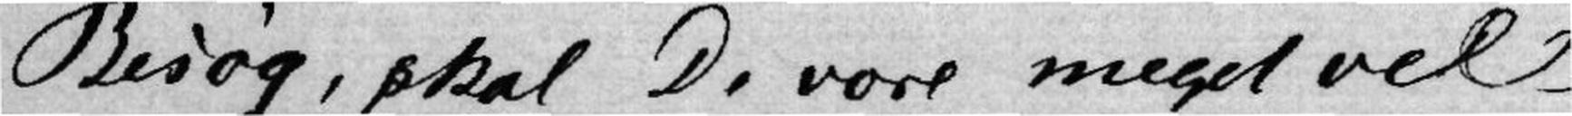Besøg, skal De være meget vel-
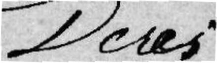Deres
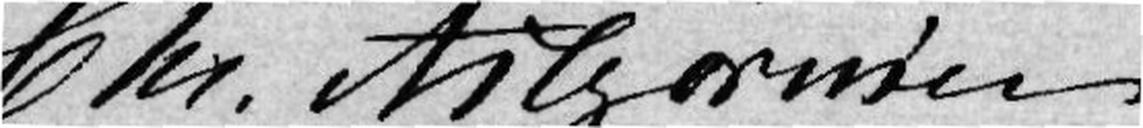P. Chr. Asbjørnsen
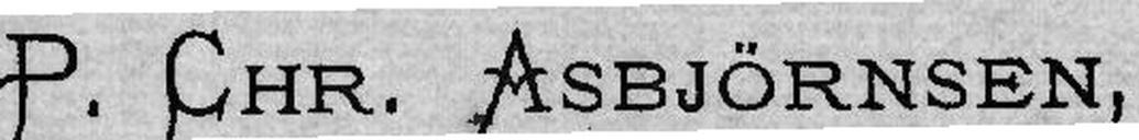P. CHR. ASBJØRNSEN,
